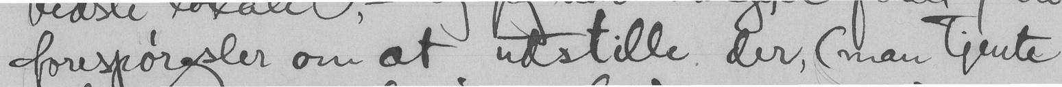forespørgsler om at udstille der, (man tjente
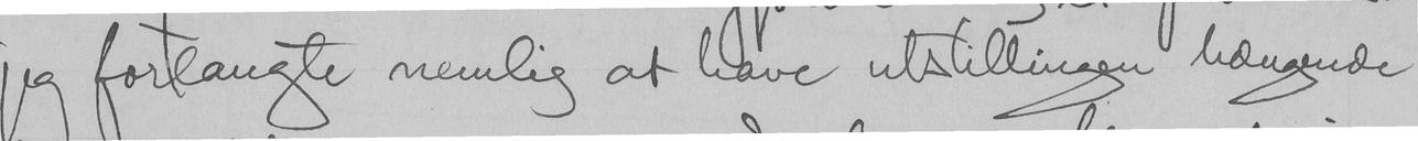jeg forlangte nemlig at have utstillingen hængende
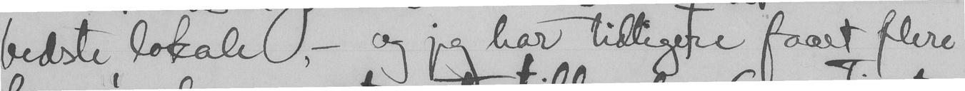bedste lokale, - og jeg har tidligere faaet flere
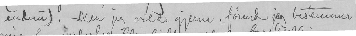endnu). Men jeg vilde gjerne, førend jeg bestemmer
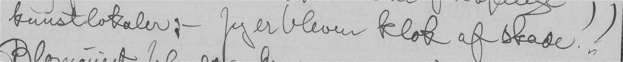kunstlokaler; - Jeg er bleven klok af skade!!
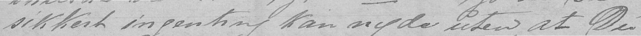sikkert ingenting kan nyde uten at Du
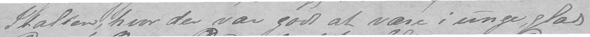Italien, hvor der var godt at være i unge glade
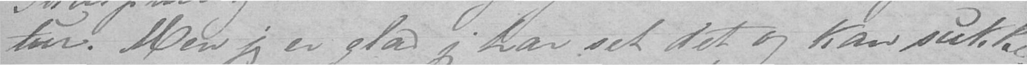tur. Men jeg er glad jeg har set det og kan sukke.
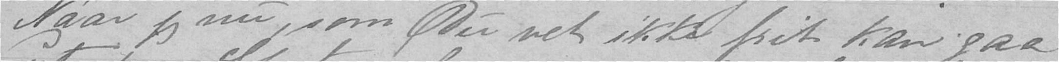Naar jeg nu, som Du vet ikke frit kan gaa
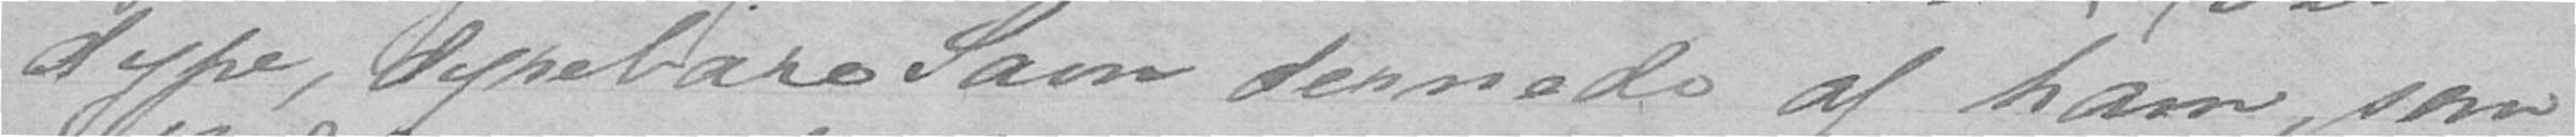dype, dyrebare Savn dernede af ham, som
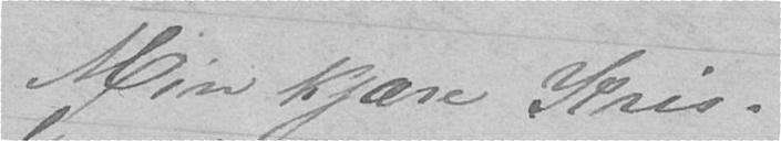Min Kjære Kris
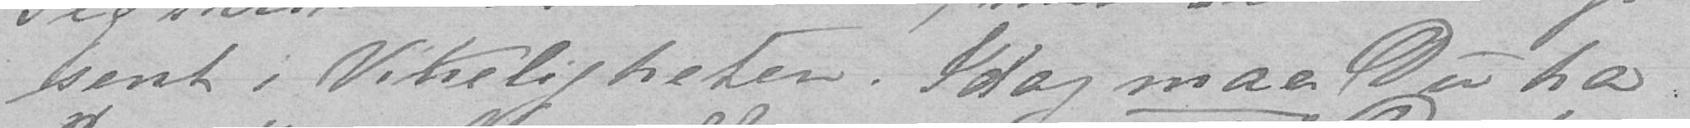sent i Virkeligheten. Idag maa Du ha
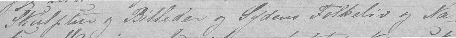Skulptur og Billeder og Sydens Folkeliv og Na-
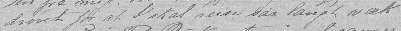drøvet for at I skal reise saa langt væk
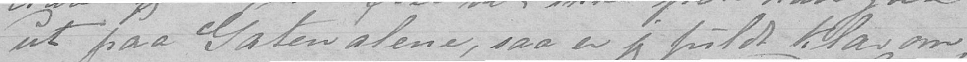ut paa Gaten alene, saa er jeg fuldt klar om,
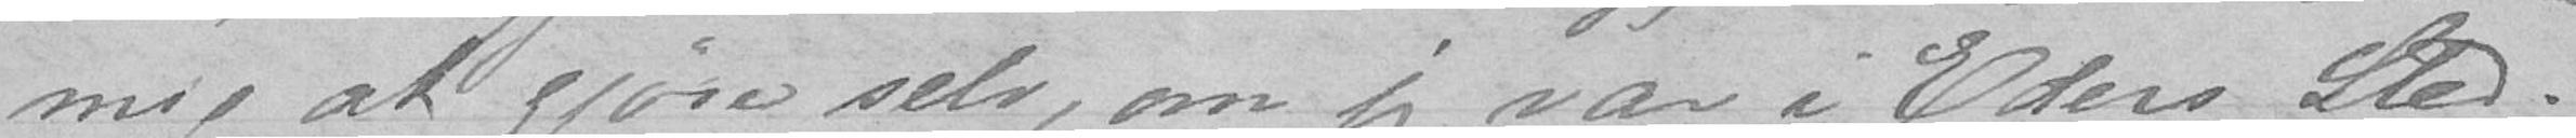mig at gjøre selv, om jeg var i Eders Sted.
